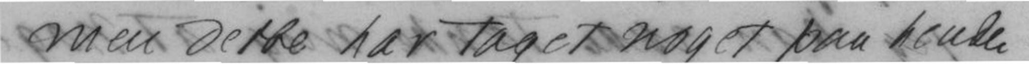men Dette har taget noget paa hende.
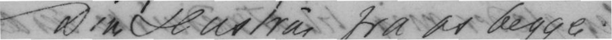Din Hustru fra os begge.
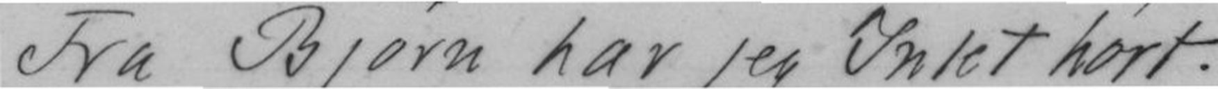Fra Bjørn har jeg Intet hørt
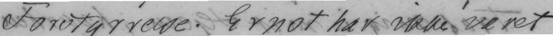Forstyrrelse. Ernst har ikke været
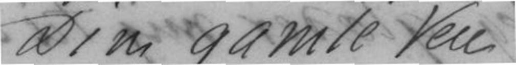Din gamle Ven
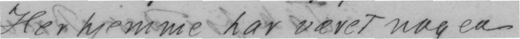Her hjemme har været nogen
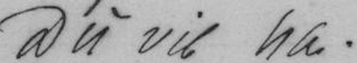Du vil ha.
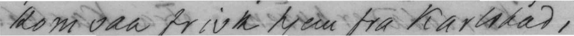kom saa frisk hjem fra Karlstad
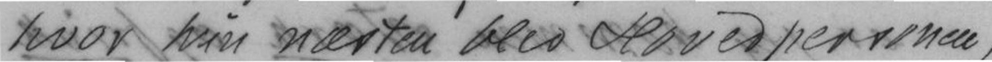hvor hun næsten blev hovedpersonen,
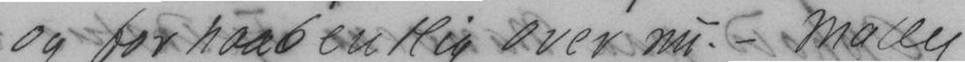og forhaabentlig over nu. Mally
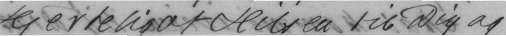Hjerteligst Hilsen til Dig og
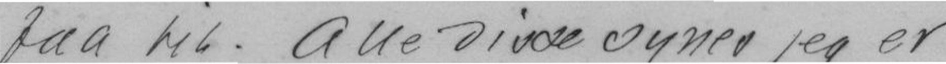faa til. Alle disse synes jeg er
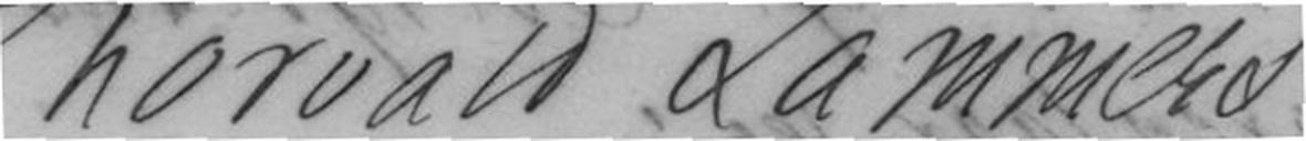Thorvald Lammers
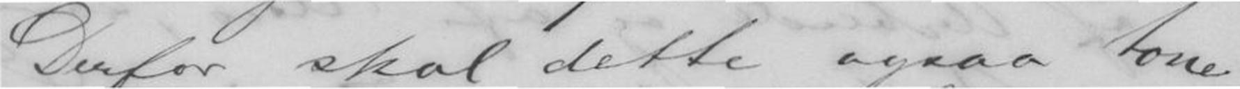Derfor skal dette ogsaa tone,
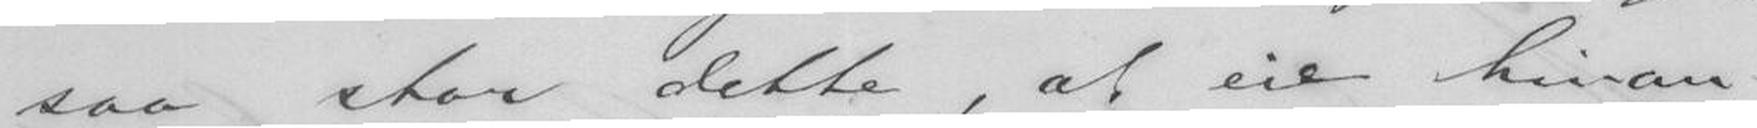saa stor dette, at eie hinan-
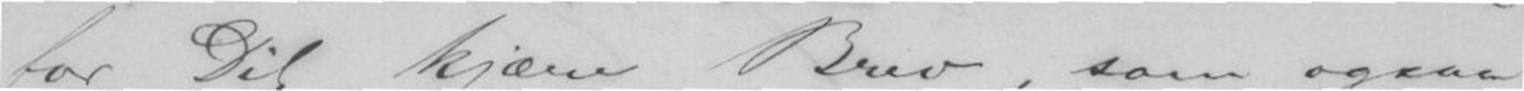for Dit kjære Brev, som ogsaa
